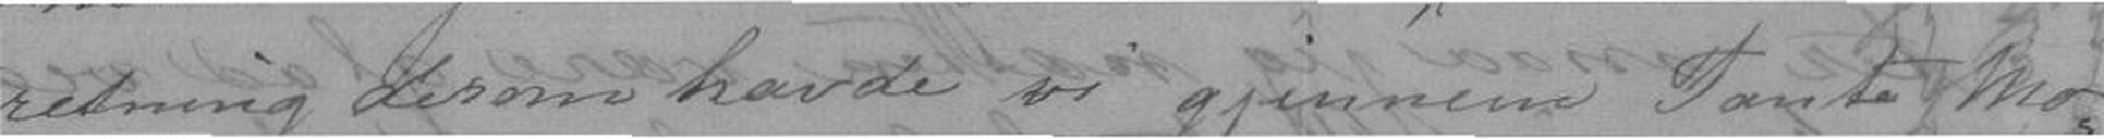retning derom havde vi gjennem Tante Mo-
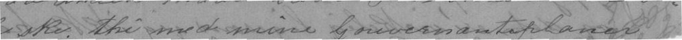le ske; thi med mine Gouvernanteplaner
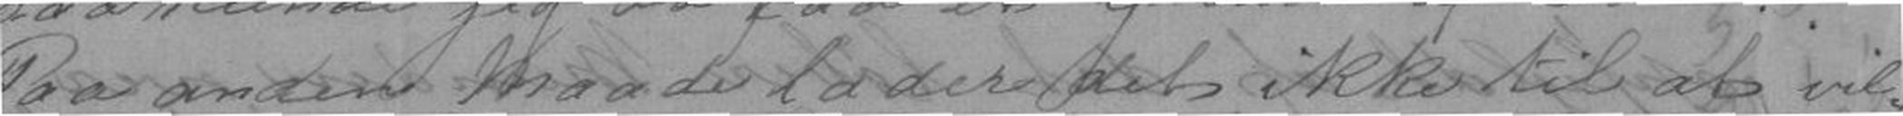Paa anden Maade lader det ikke til at vil-
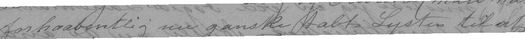forhaabentlig nu ganske tabt Lysten til at
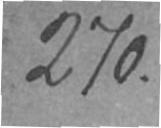270.
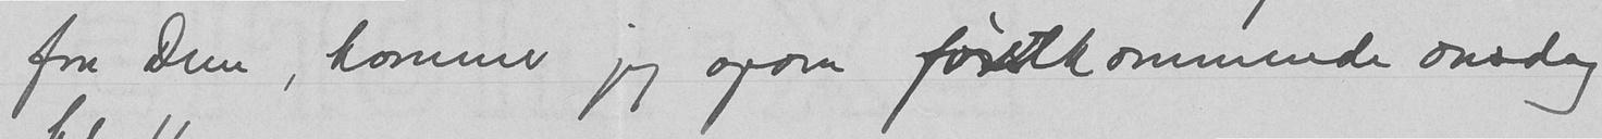fra Dem, kommer jeg ogsaa førstkommende onsdag
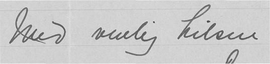Med venlig hilsen
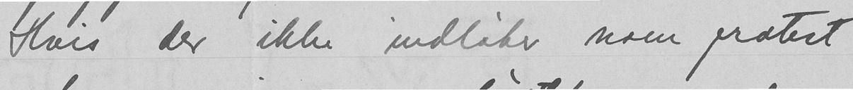Hvis der ikke indløber noen protest
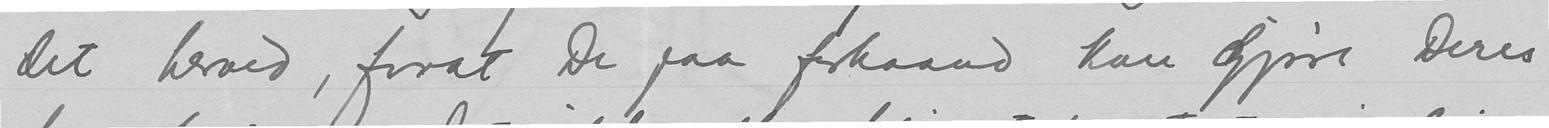det herved, forat De paa forhaand kan gjøre Deres
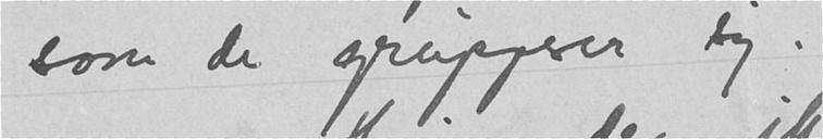som de grupperer sig.
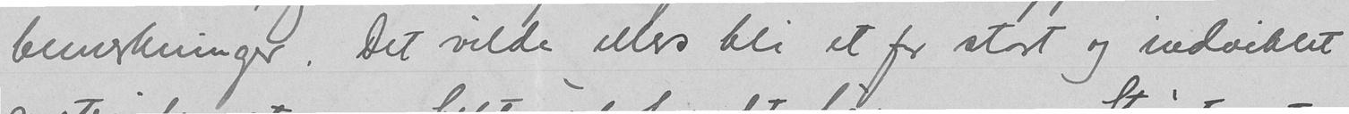bemerkninger. Det vilde ellers bli et for stort og indviklet
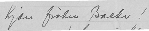Kjære frøken Backer!
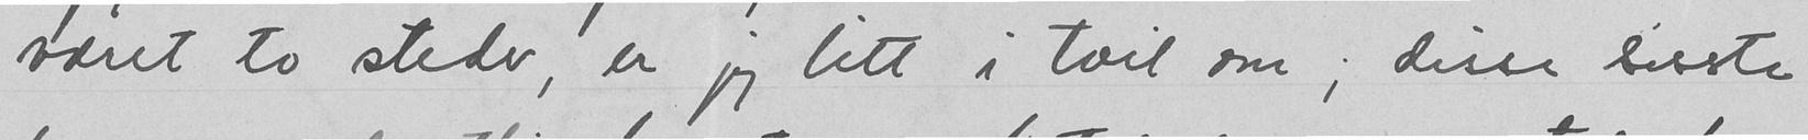været to steder, er jeg litt i tvil om; dise sisste
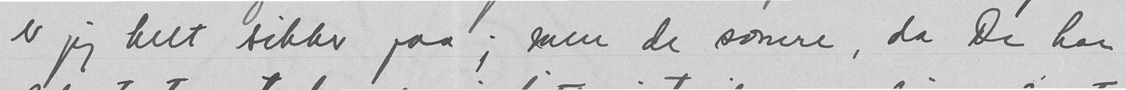er jeg helt sikker paa; men de somre, da De har
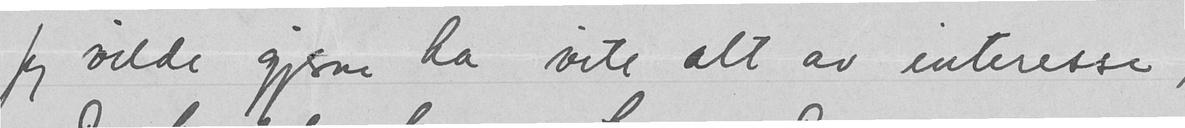Jeg vilde gjerne da vite alt av interesse,
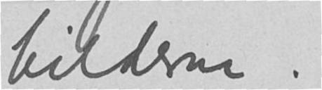bilderne.
